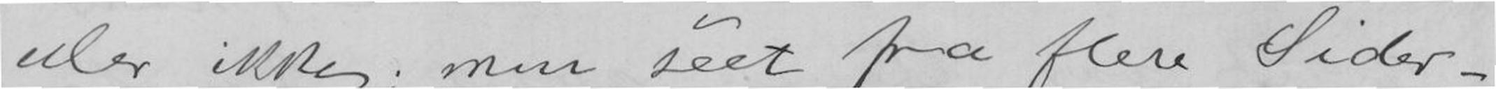eller ikke; men seet fra flere Sider -
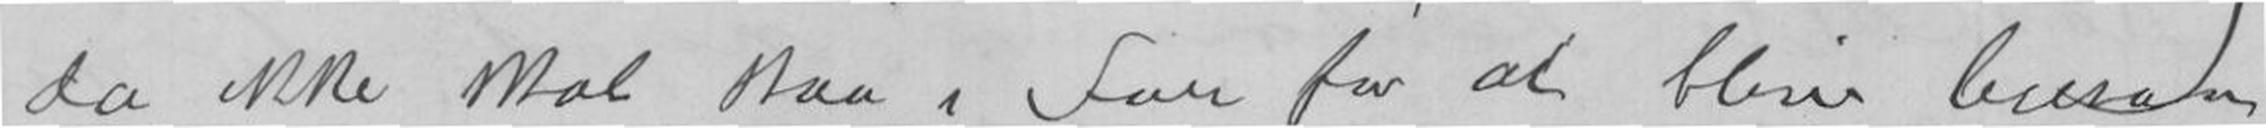da ikke skal staa i Fare for at blive Ensom
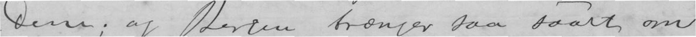Dem; og Bergen trænger saa saart om
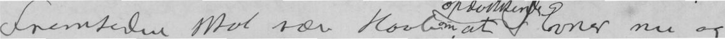Fremtiden mot skal være Haab om, at Evner nu og
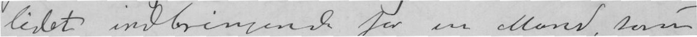lidet indbringende for en Mand, som
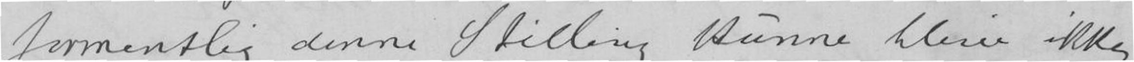formentlig denne Stilling kunne blive ikke
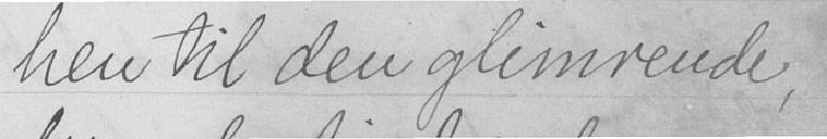hen til den glimrende,
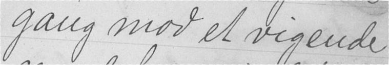gang mod et vigende
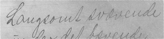Langsomt svævende
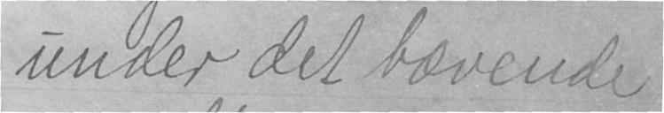under det bævende
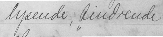lysende, tindrende
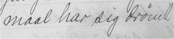maal har sig drømt
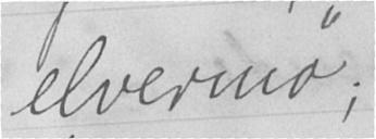elvermø;
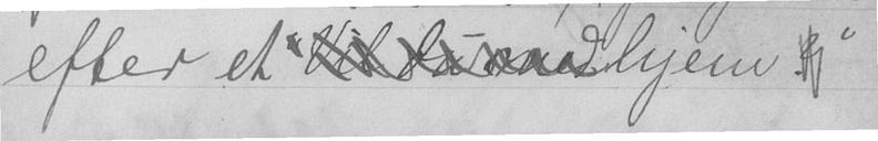efter et Vil du med hjem? "
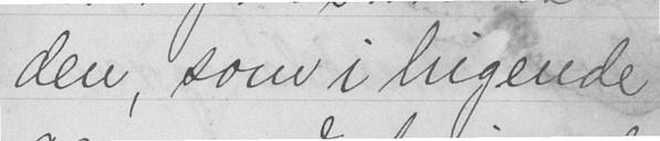den, som i higende
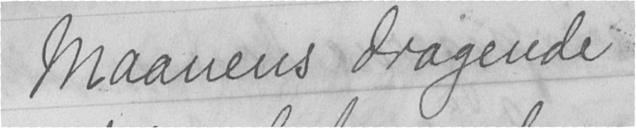Maanens dragende
